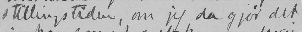stillingstiden, om jeg da gjør det,
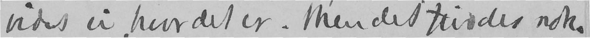vides ei, hvor det er. Men det findes nok.
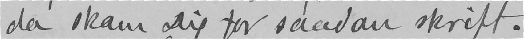da skam Dig for saadan skrift.
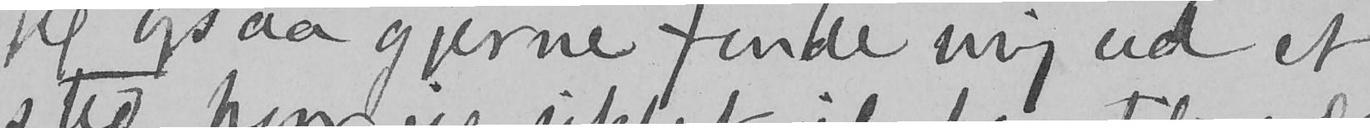jeg ogsaa gjerne finde mig ud et
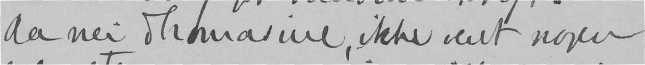Aa nei Thomasine, ikke vent nogen
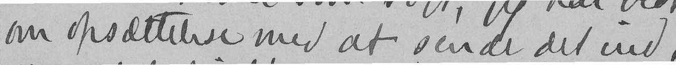om opsættelse med at sende det ind,
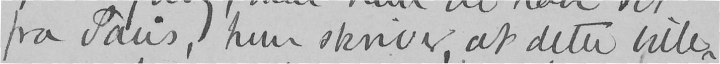fra Paris, hun skriver, at dette bille-
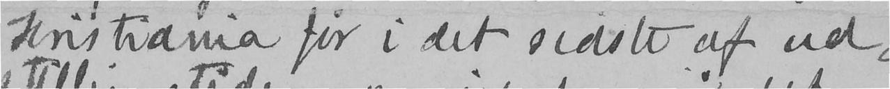Kristiania for i det sidste af ud-
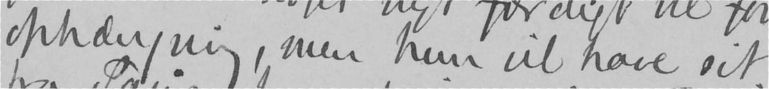ophængning, men hun vil have sit
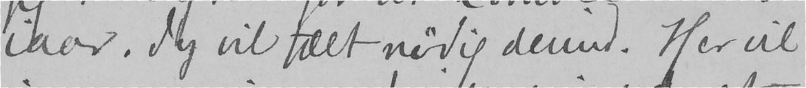iaar. Jeg vil fælt nødig derind. Her vil
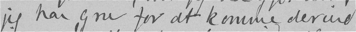jeg har gru for at komme derind
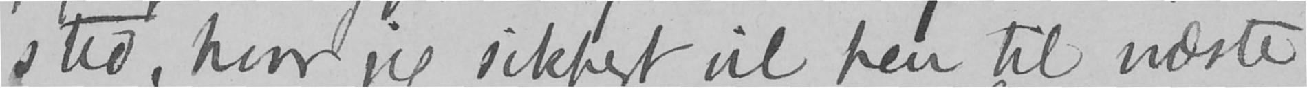sted, hvor jeg sikkert vil hen til næste
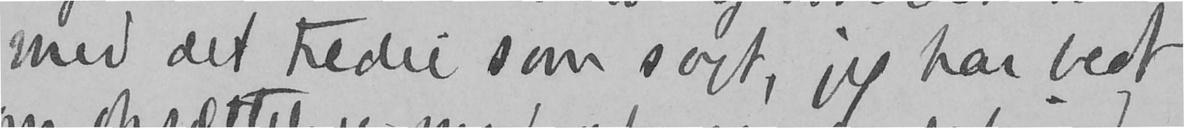med det tredie som sagt, jeg har bedt
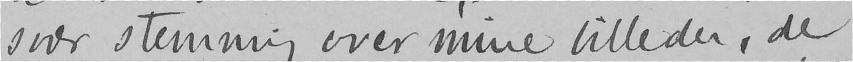svær stemning over mine billeder, de
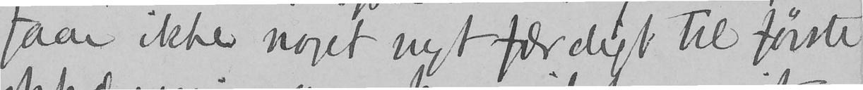faar ikke noget nyt færdigt til første
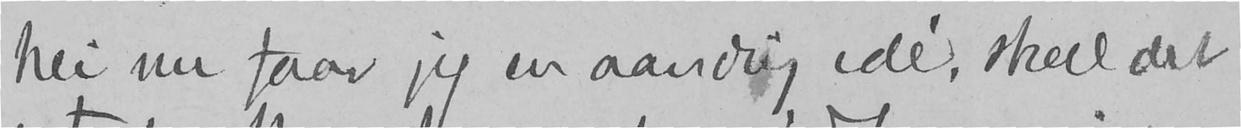Nei nu faar jeg en aandrig idé, skal det
